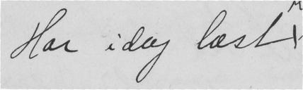Har idag læst
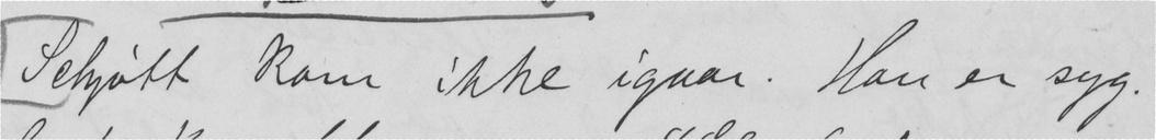Schjøtt kom ikke igaar. Han er syg.
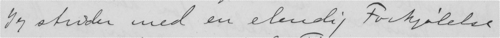Jeg strider med en elendig Forkjølelse
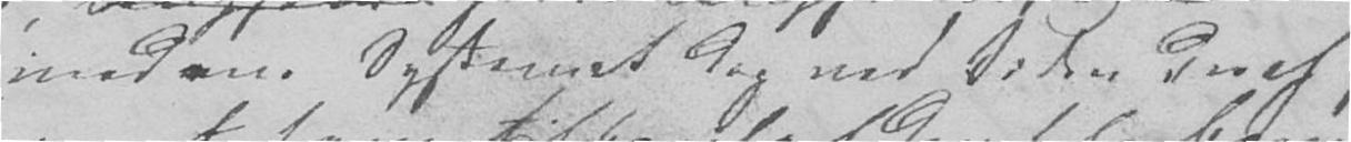medens Systemet dog ved Siden deraf
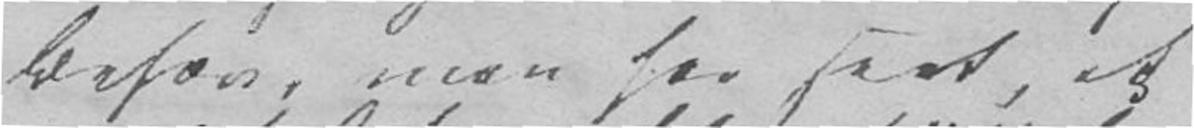Behov, man har seet, at
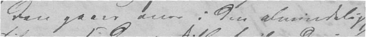Den gaaer over i den almindelig
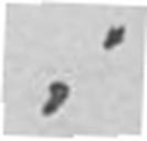i
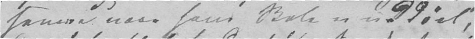senere naar hans Skole er uddøet,
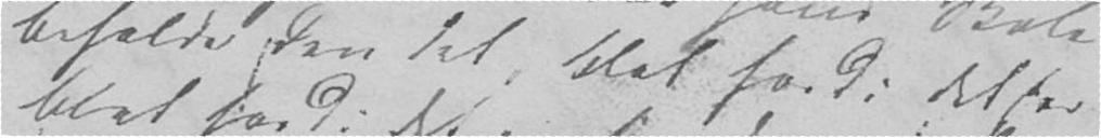beholder den det, blot fordi det har
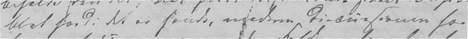blot fordi det er sandt, medens Discussionen har
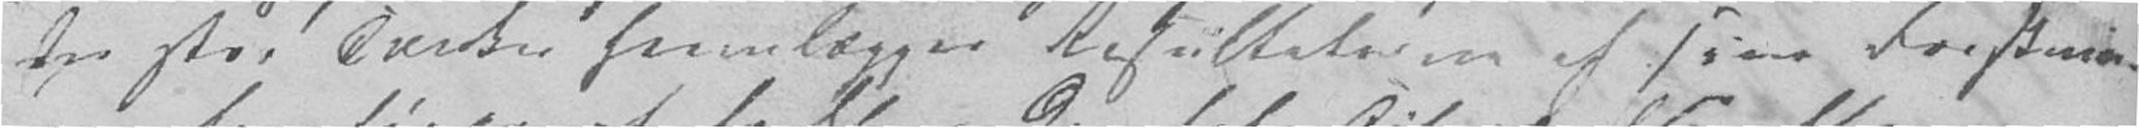En stor Tænker fremlægger Resultaterne af sine Forsknin-
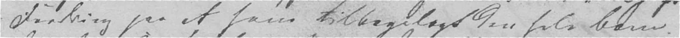Fordring paa at have tilbagelagt den hele Bane.
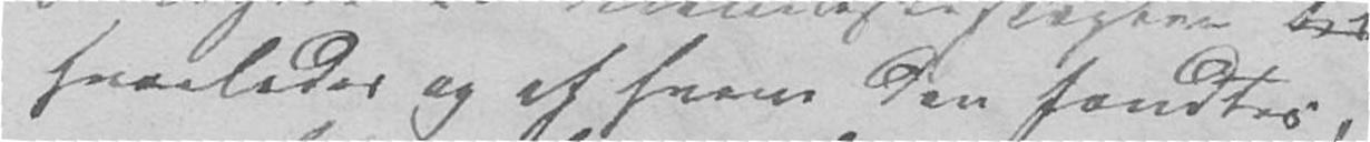hvorledes og af hvem den fandtes,
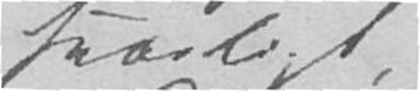sværligt,
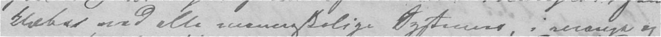klæber ved alle menneskelige Systemer, i mange og
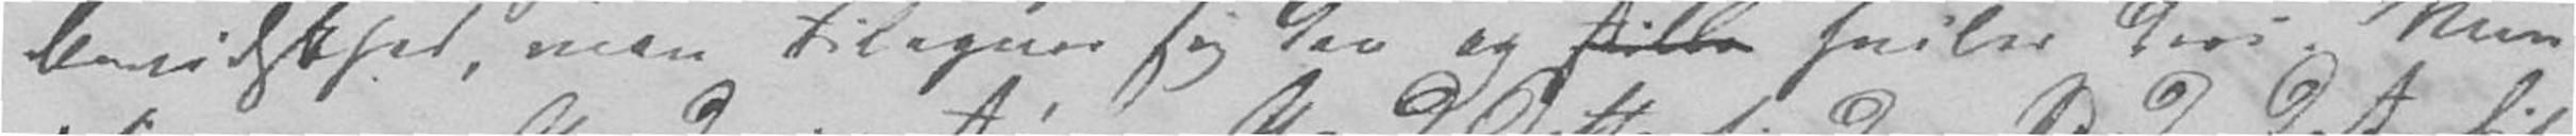Bevidsthed, man tilegner sig den og hviler deri. Men
